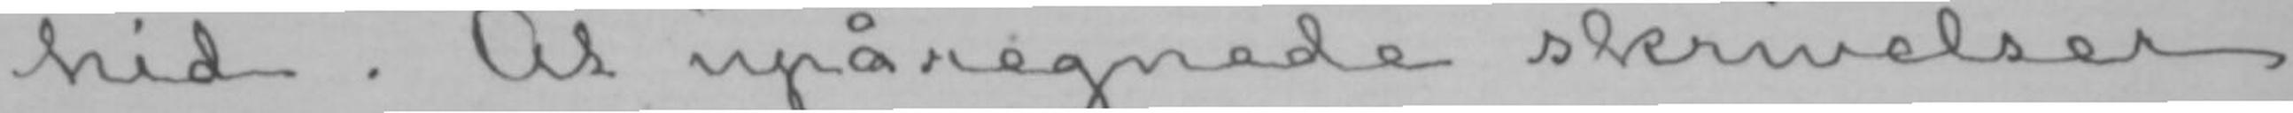hid. At upåregnede skrivelser
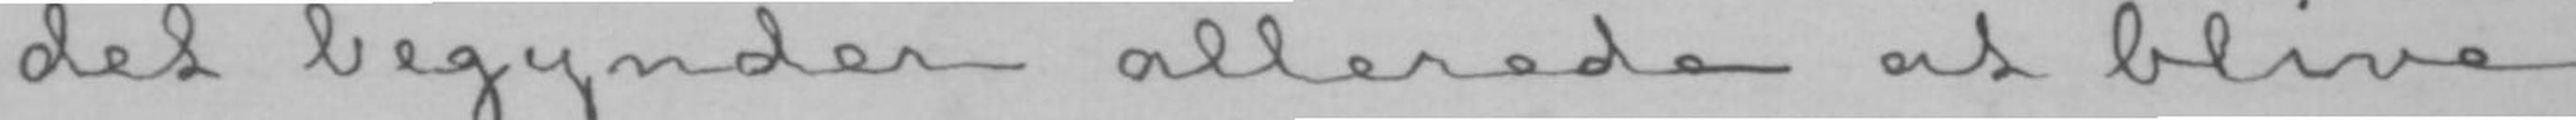det begynder allerede at blive
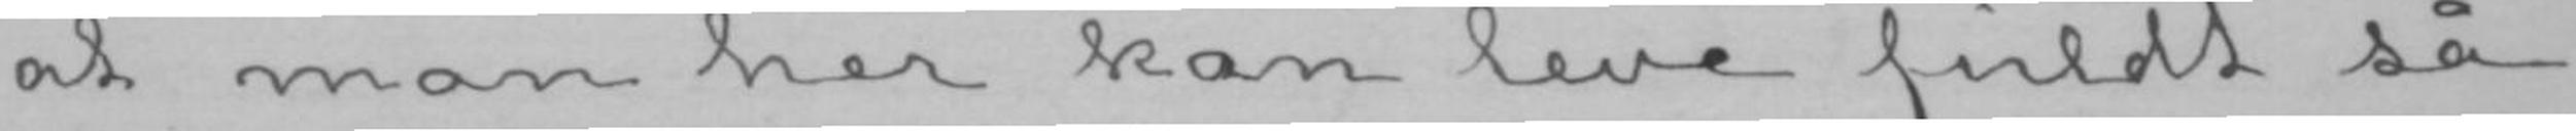at man her kan leve fuldt så
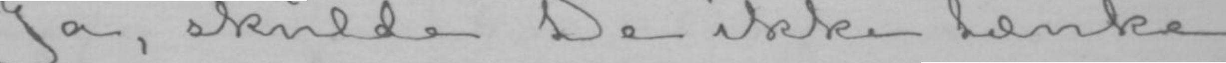Ja, skulde De ikke tænke
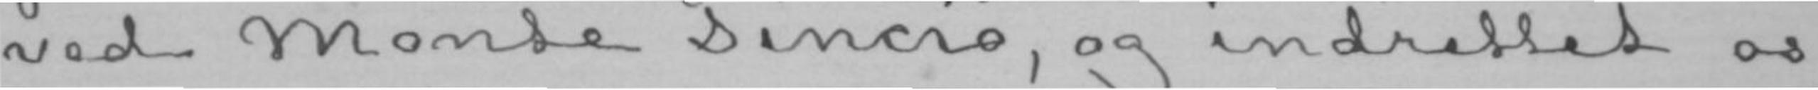ved Monte Pincio, og indrettet os
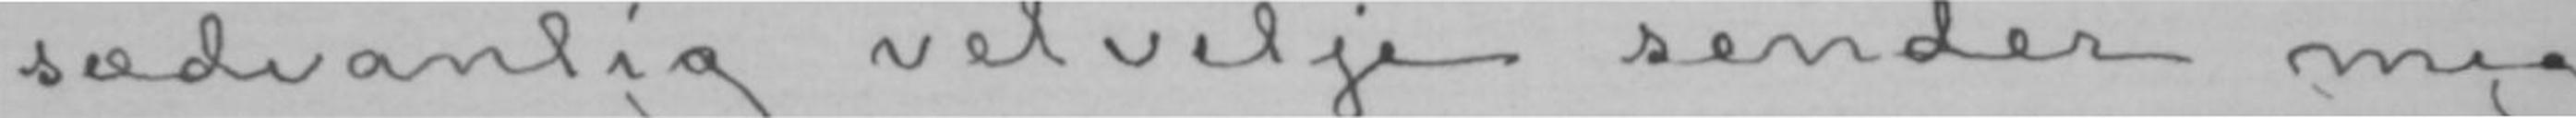sædvanlig velvilje sender mig
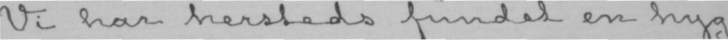Vi har hersteds fundet en hyg-
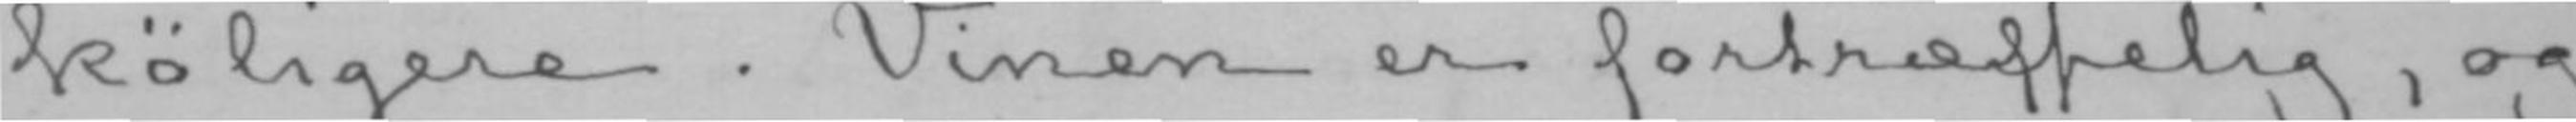køligere. Vinen er fortræffelig, og
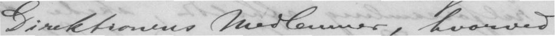Direktionens Medlemmer, hvorved
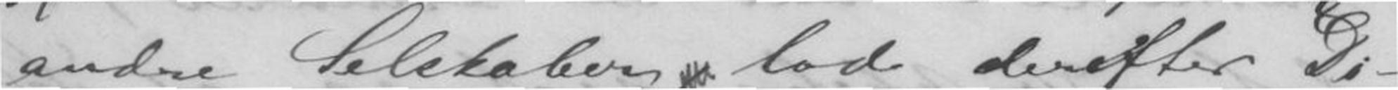andre Selskaber , lod derefter Di-
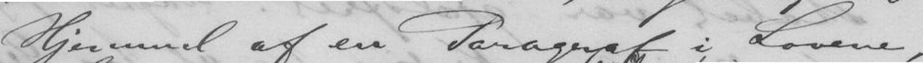Hjemmel af en Paragraf i Lovene,
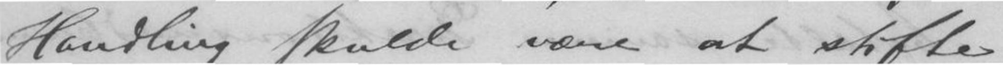Handling skulde være at stifte
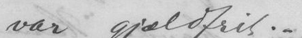var gjældfrit.
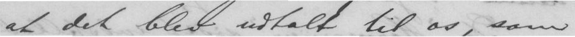at det blev udtalt til os, som
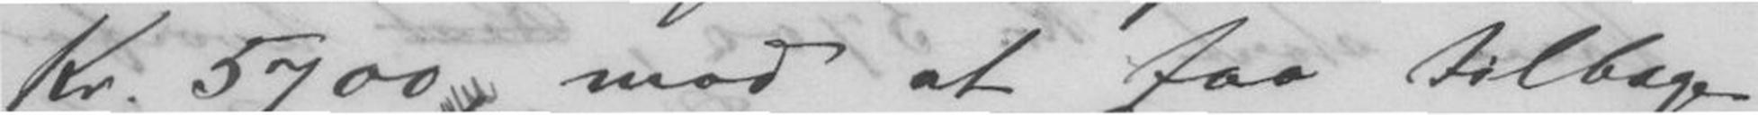Kr. 5700, mod at faa tilbage
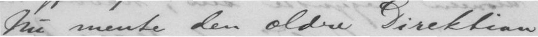Nu mente den ældre Direktion
